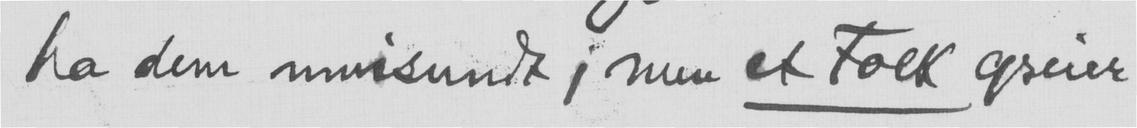ha dem missundt; men et Folk greier
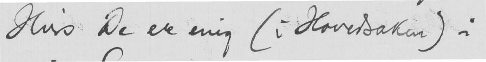Hvis De er enig (i Hovedsaken) i
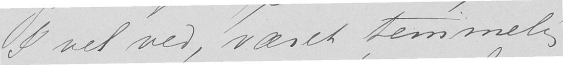I vel ved, været temmelig
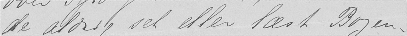de aldrig set eller læst Bogen.
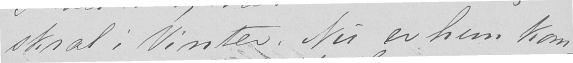skral i Vinter. Nu er hun kom-
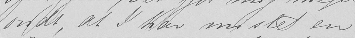ondt, at I har mistet en
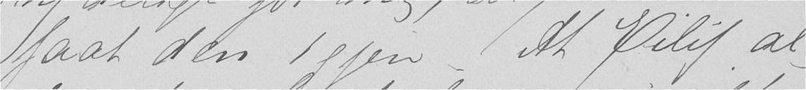faat den igjen. At Eilif al-
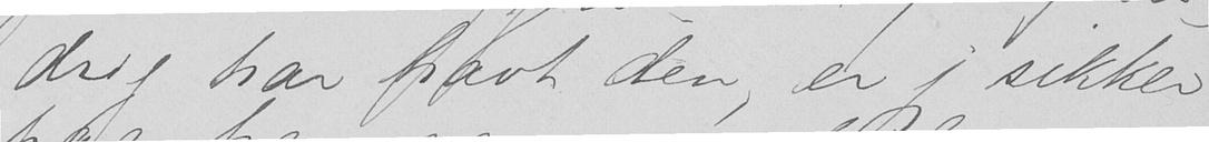drig har havt den, er jeg sikker
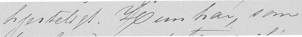hjerteligt. Hun har, som
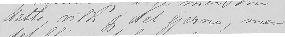dette, vilde jeg det gjerne; men
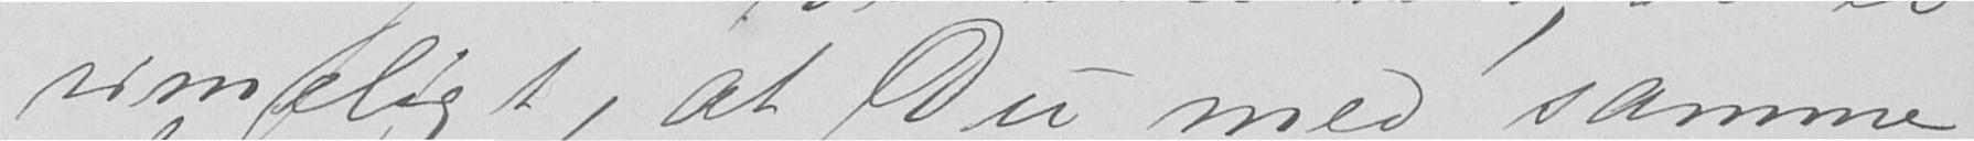rimeligt, at Du med samme
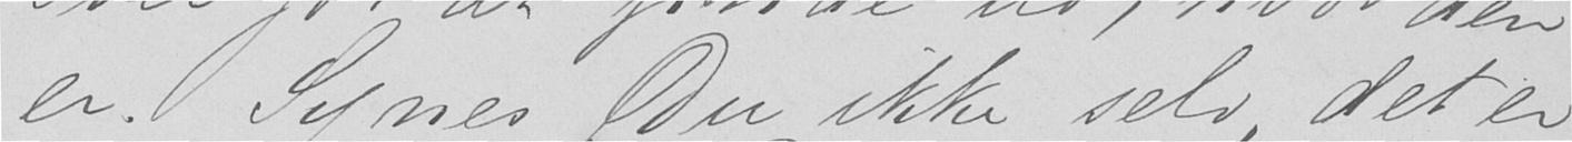er. Synes Du ikke selv det er
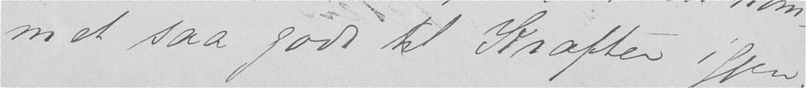met saa godt til Kræfter igjen,
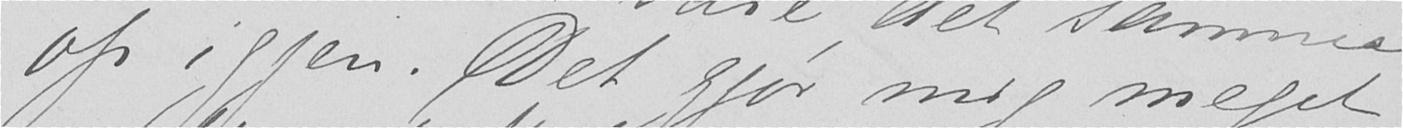op igjen. Det gjør mig meget
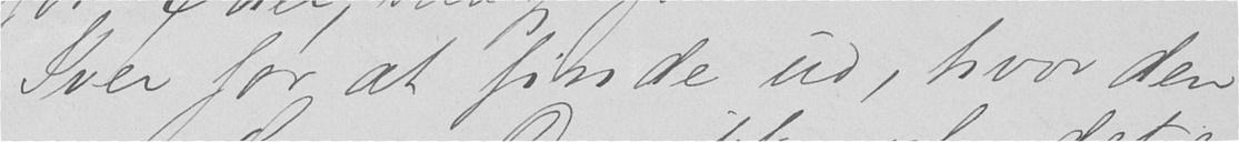Iver for at finde ud, hvor den
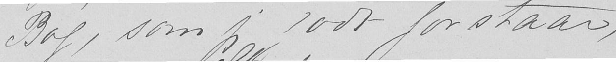Bog, som jeg godt forstaar,
